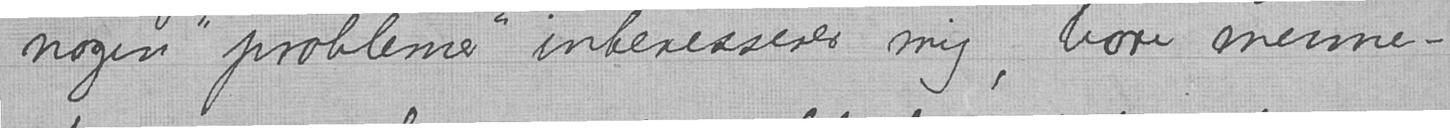nogen "problemer" interesserer mig, bare menne-
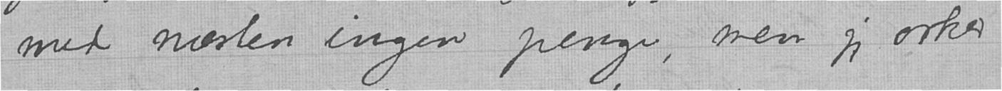med næsten ingen penge, men jeg orker
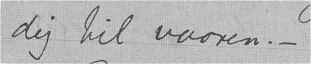dig til vaaren. -
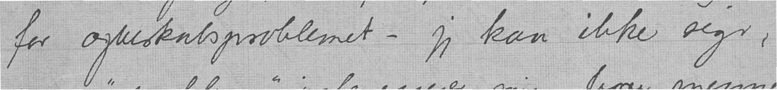for ægteskabsproblemet - jeg kan ikke sige,
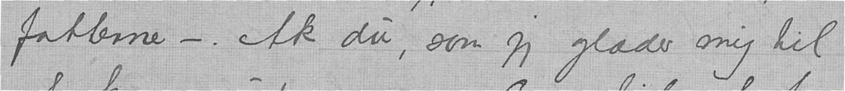fatterne -. Ak du, som jeg glæder mig til
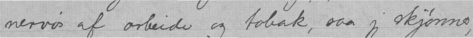nervøs af arbeide og tobak, saa jeg skjønner,
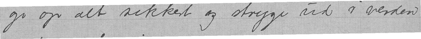gi op alt sikkert og stryge ud i verden
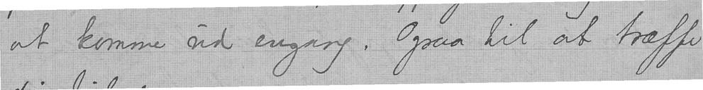at komme ud engang. Ogsaa til at træffe
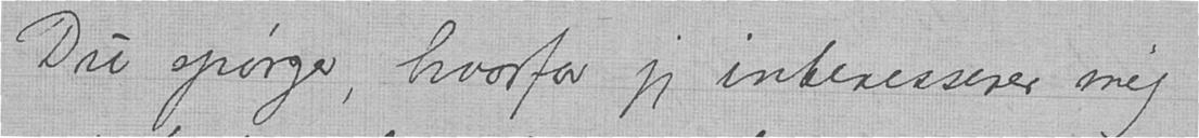Du spørger, hvorfor jeg interesserer mig
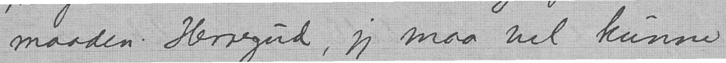maaden. Herregud, jeg maa vel kunne
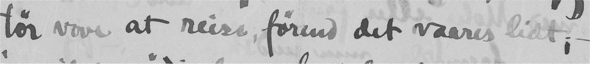tør vove at reise, førend det vaares lidt; -
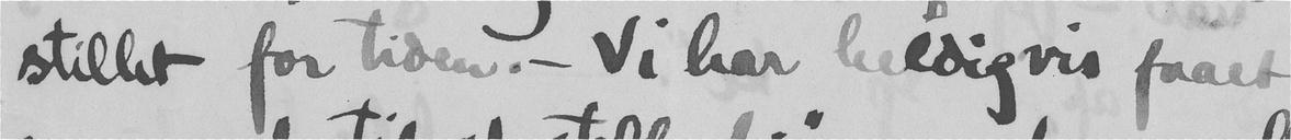stillet for tiden. - Vi har heldigvis faaet
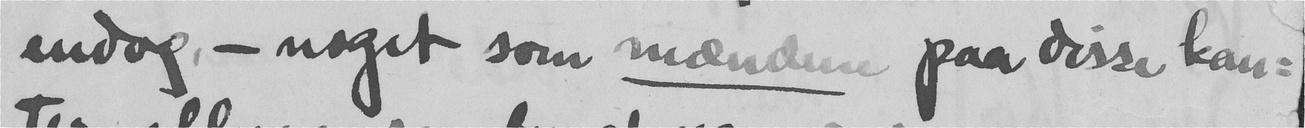endog - noget som mændem paa disse kan-
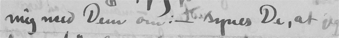mig med Dem om: -- synes De, at jeg
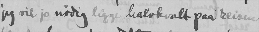jeg vil jo nødig ligge halvkvalt paa reisen
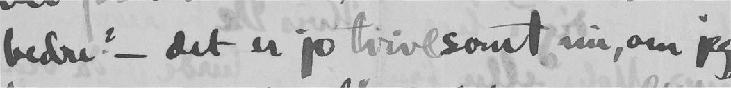bedre? - det er jo tvivlsomt nu, om jeg
